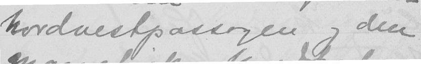Nordvestpassagen og den
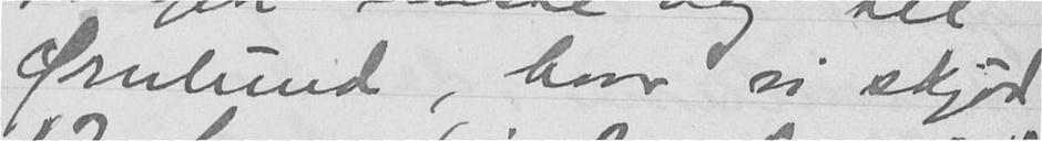Ørnlund, hvor vi skjød
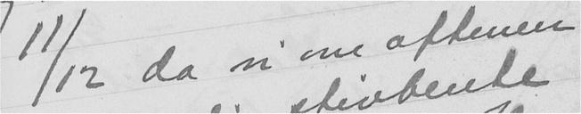11/12 da vi om aftenen
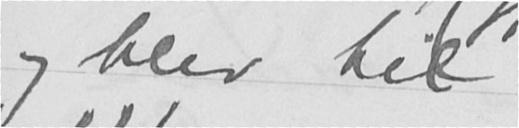og blev til
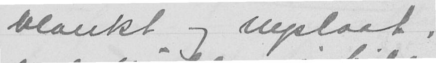blankt og nyslaat,
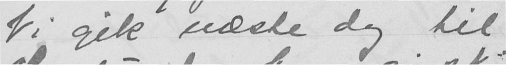Vi gik næste dag til
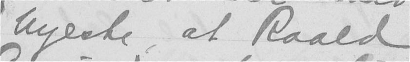Nyeste at Roald
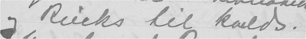og Riecks til kvelds.
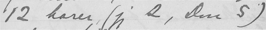12 harer (jeg 2, Don 5)
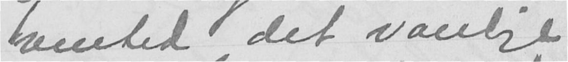vented det vanlige
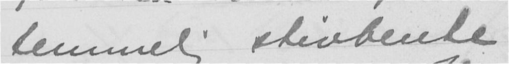temmelig stivbente
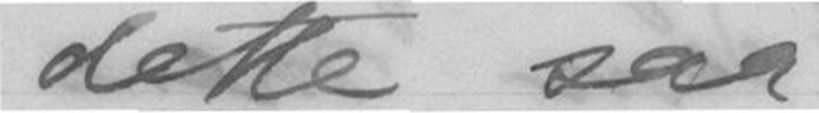dette saa
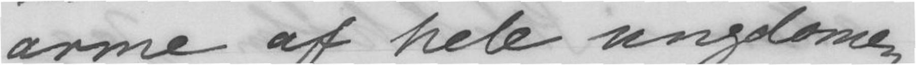arme af hele ungdomen
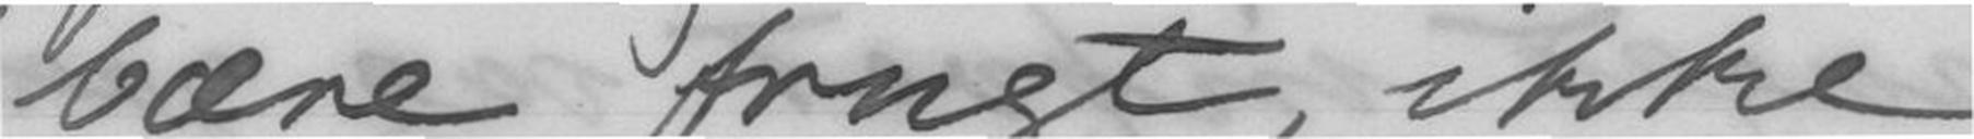bære frugt, ikke
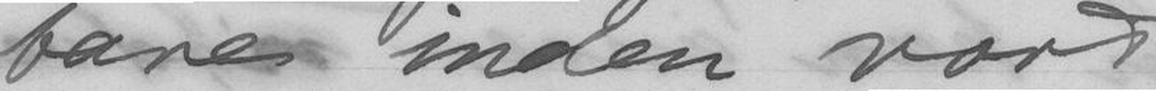bare inden vort
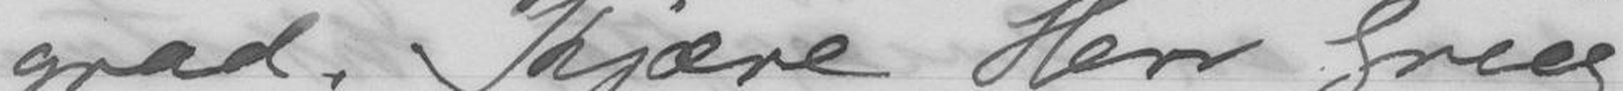grad. Kjære Herr Grieg
